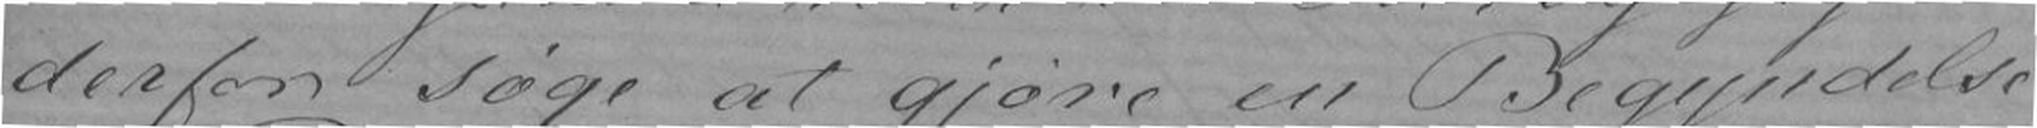derfor søge at gjøre en Begyndelse
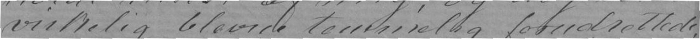virkelig blevne temmelig forudrettede
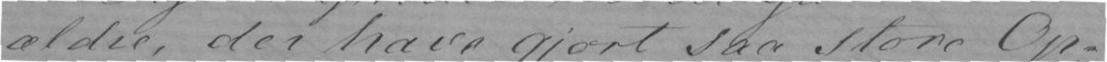ældre, der have gjort saa store Op-
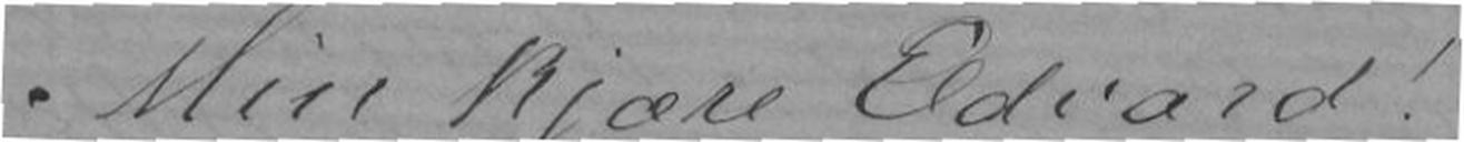Min kjære Edvard!
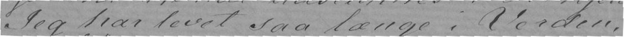Jeg har levet saa længe i Verden,
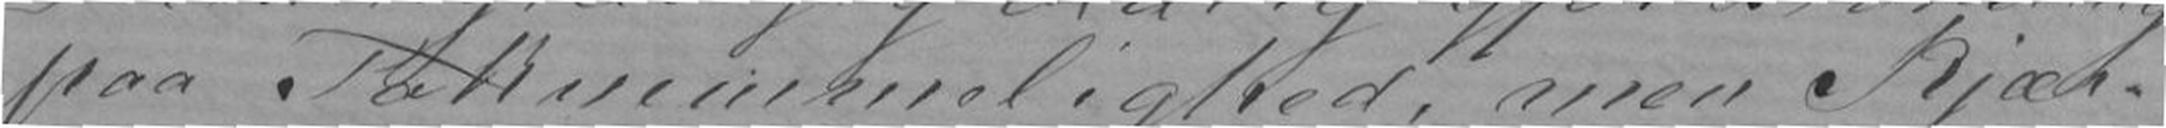paa Taknemmelighed, men Kjær-
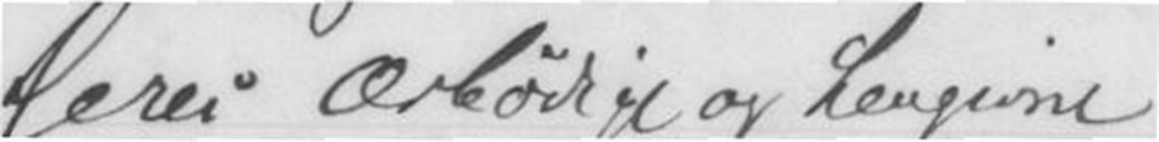Deres Ærbødige og hengivne
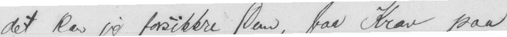det kan jeg forsikre Dem, faa Krav paa
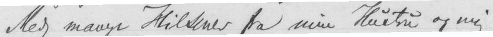Med mange Hilsener fra min Hustru og mig
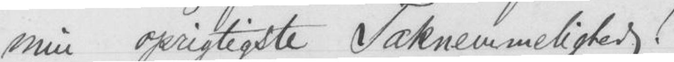min oprigtigste Taknemmelighed !
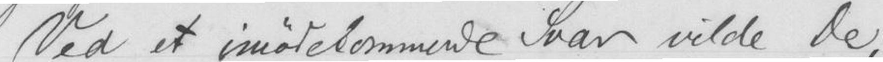Ved et imødekommende Svar vilde De,
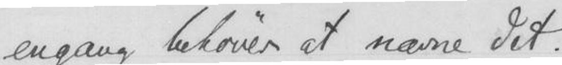engang behøver at nævne det.
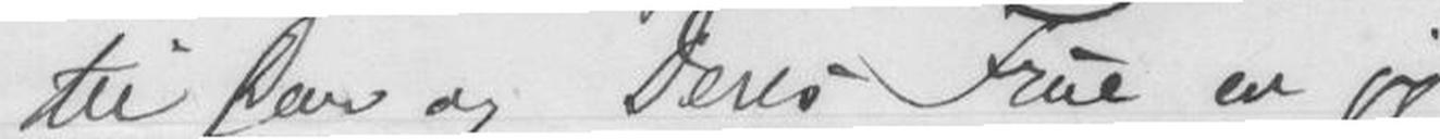til Dem og Deres Frue er jeg
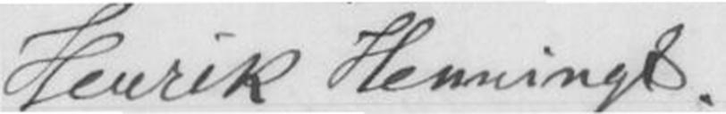Henrik Hennings.
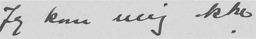Jeg kom mig ikke
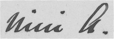Nini A.
